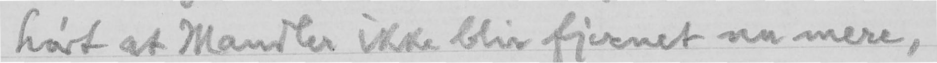hørt at Mandler ikke blir fjernet nu mere,
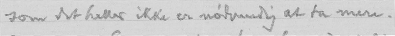som det heller ikke er nødvendig at ta mere.
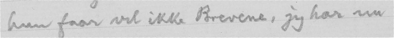hun faar vel ikke Brevene, jeg har nu
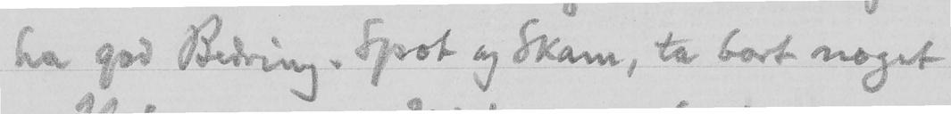ha god Bedring. Spot og Skam, ta bort noget
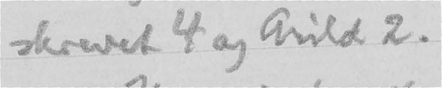skrevet 4 og Arild 2.
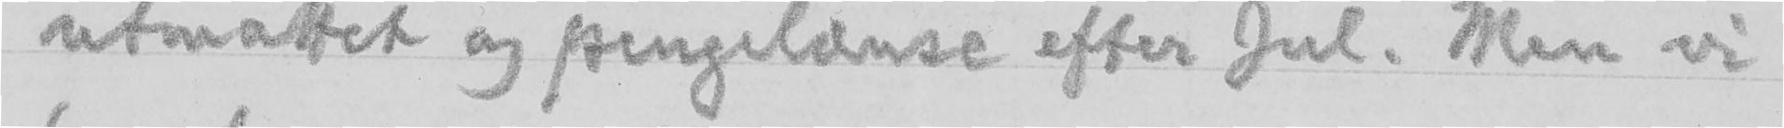utmattet og pengelænse efter Jul. Men vi
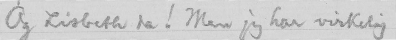Og Lisbeth da! Men jeg har virkelig
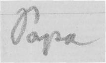Papa
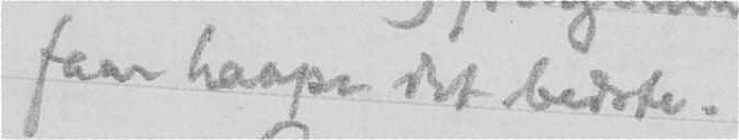faar haape det bedste.
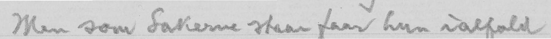Men som Sakerne staar faar hun ialfald
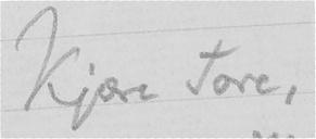Kjære Tore,
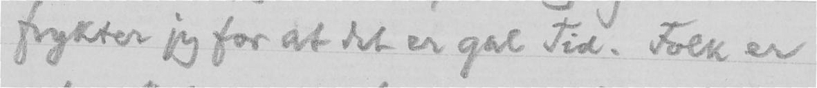frykter jeg for at det er gal Tid. Folk er
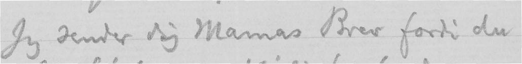Jeg sender dig Mamas Brev fordi du
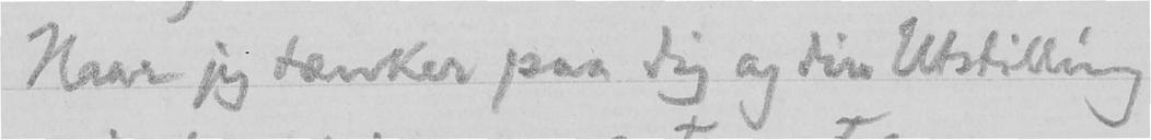Naar jeg tænker paa dig og din Utstilling
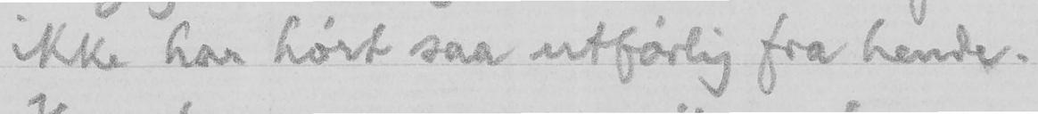ikke har hørt saa utførlig fra hende.
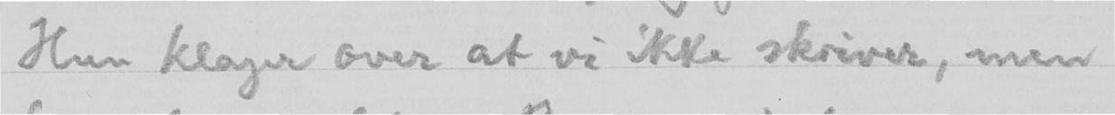Hun klager over at vi ikke skriver, men
